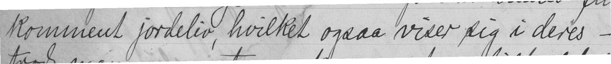komment jordeliv, hvilket ogsaa viser sig i deres -
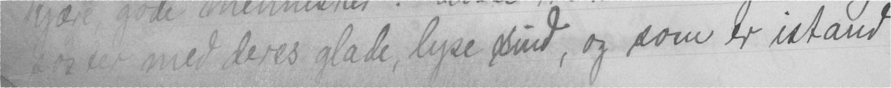søster med deres glade, lyse sind, og som er istand
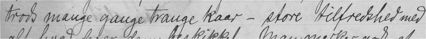trods mange gange trange kaar - store tilfredshed med
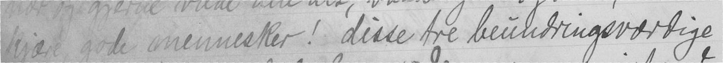kjære, gode mennesker! disse tre beundringsværdige
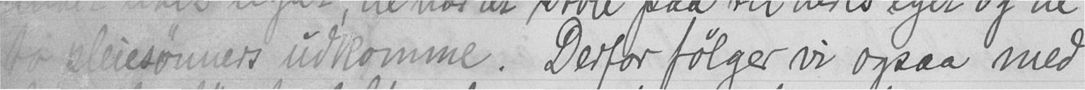to pleiesønners udkomme. Derfor følger vi ogsaa med
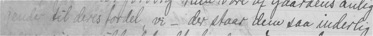gender til deres fordel, vi - der staar dem saa inderlig
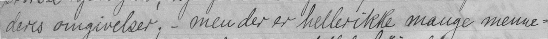deres omgivelser; - men der er hellerikke mange menne-
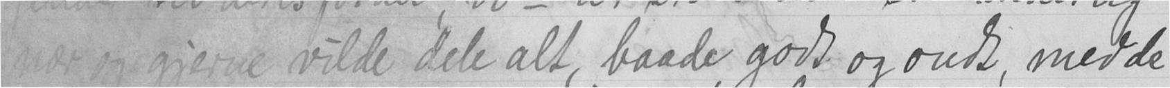nær og gjerne vilde dele alt, baade godt og ondt, med de
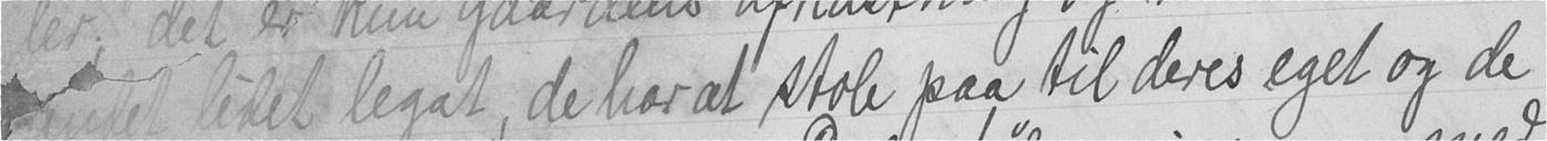andet lidet legat, de har at stole paa til deres eget og de
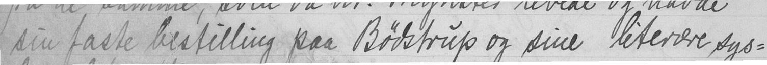sin faste bestilling paa Bødstrup og sine, literære sys-
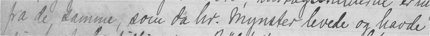fra de samme, som da hr. Mynster levede og havde
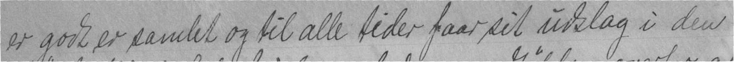er godt er samlet og til alle tider faar sit udslag i den
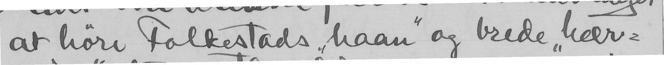at høre Folkestads "haan" og brede "hær-
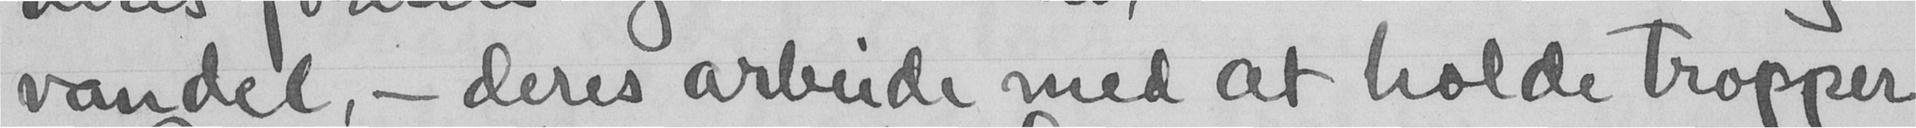vandel, - deres arbeide med at holde tropper
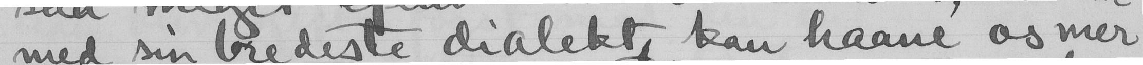med sin bredeste dialekt kan haane os mer
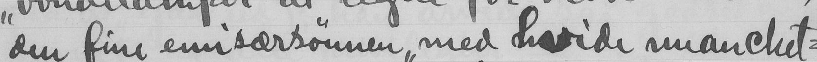den fine emisærsønnen "med hvide manchet-
